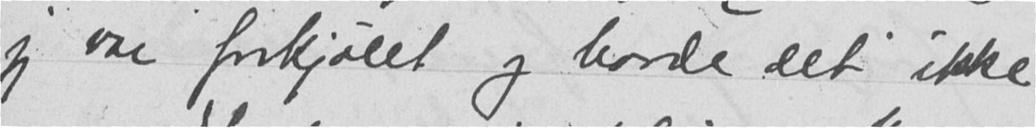jeg var forkjølet og havde det ikke
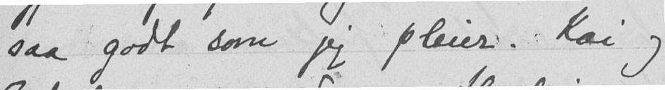saa godt som jeg pleier. Kai og
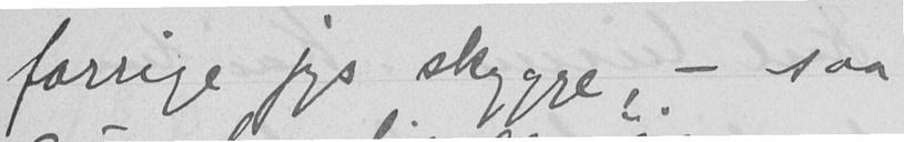forrige jegs skygge, - saa
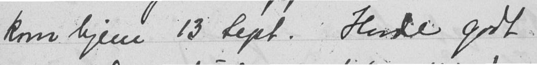kom hjem 13 Sept. Havde godt
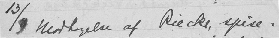13/9 Modtagelse af Riecks, spise-
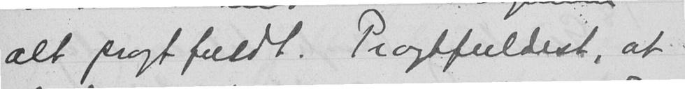alt pragtfuldt. Pragtfuldest, at
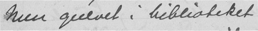Men gulvet i biblioteket
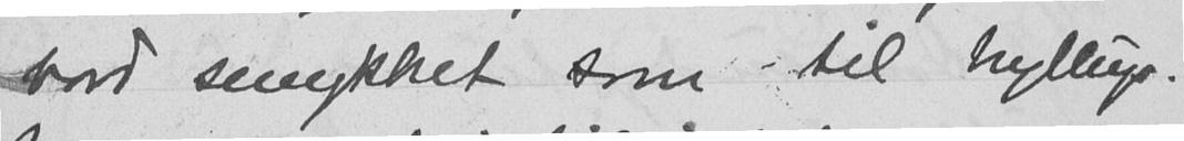bord smykket som til bryllup.
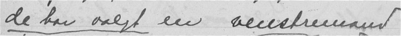de har valgt en venstremand
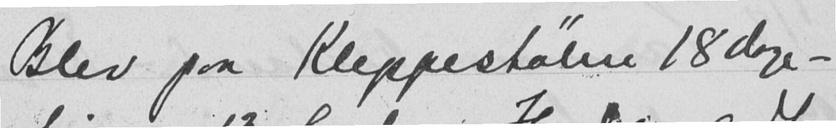Blev paa Kleppestølen 18 dage -
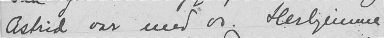Astrid var med os. Herhjemme
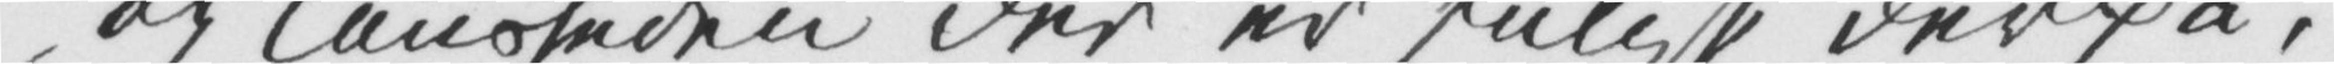og Tausheden der er fulgt derpå,
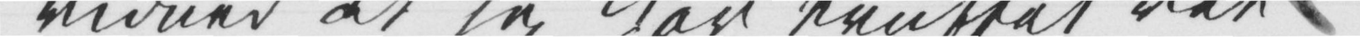vidner at jeg har truffet ret.
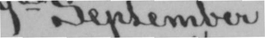19de September
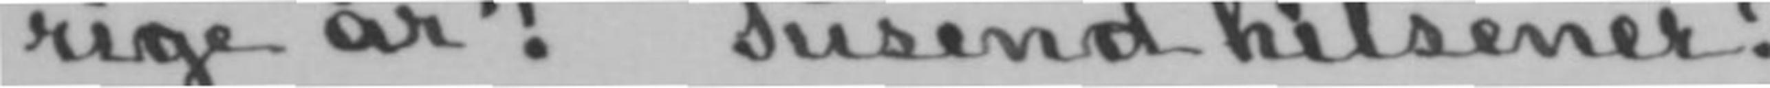rige år! Tusend hilsener!
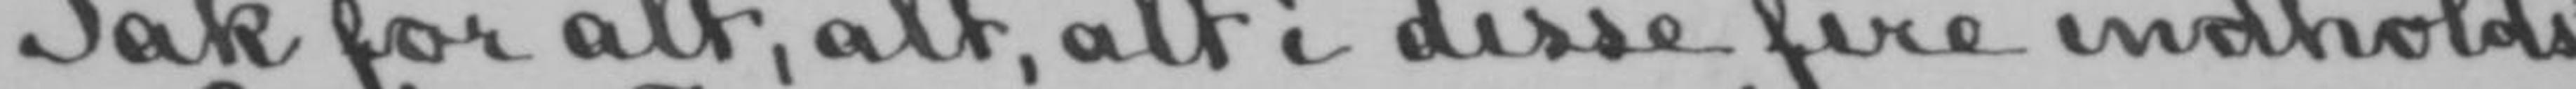Tak for alt, alt, alt i disse fire indholds-
